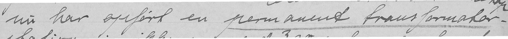nu har opført en permanent transformator-
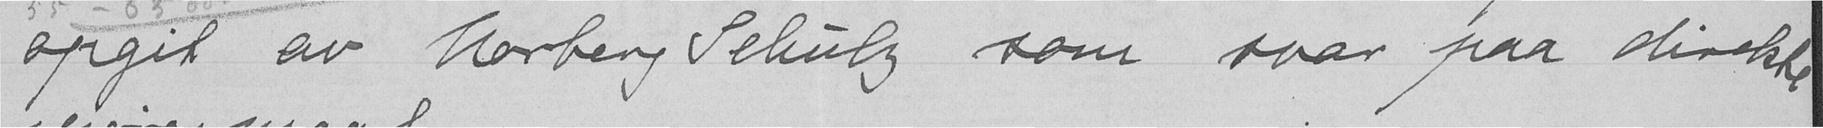opgit av Norberg Schulz som svar paa direkte
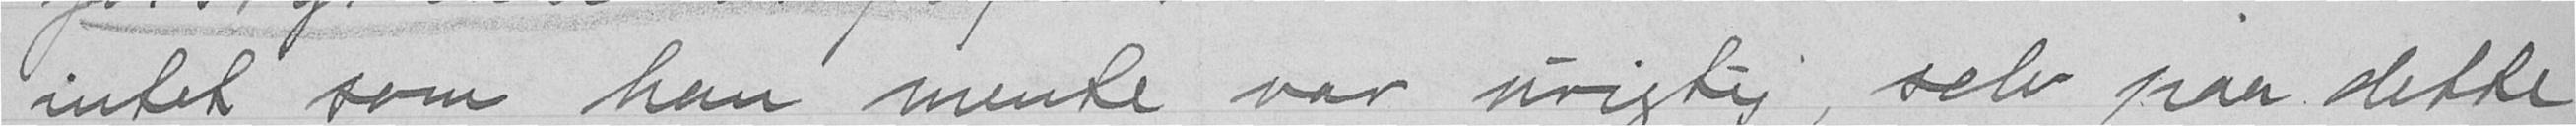intet som han mente var urigtig, selv paa dette
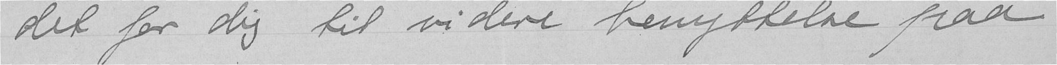det for dig til videre benyttelse paa
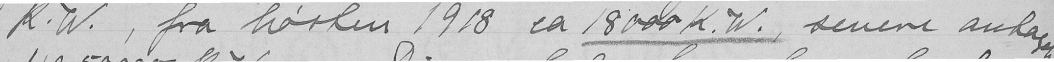K.W., fra høsten 1918 ca 18000 K.W. senere antagelig
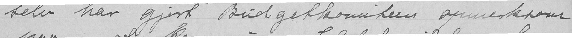selv har gjort Budgetkomiteen opmerksom
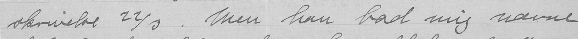skrivelse 22/3. Men han bad mig nævne
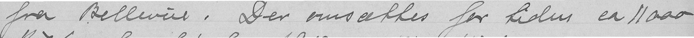fra Bellevue. Der omsættes for tiden ca 11000
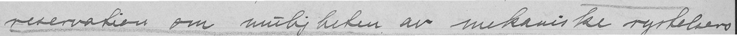reservation om muligheden av mekaniske rystelsers
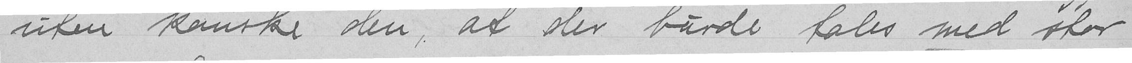uten kanske den, at der burde tales med stor
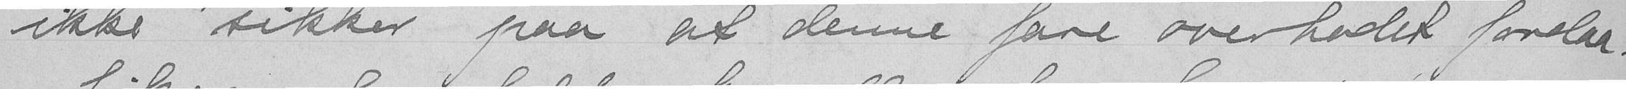ikke sikker paa at denne fare overhodet forelaa.
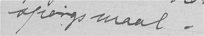spørgsmaal.
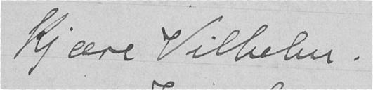Kjære Vilhelm!
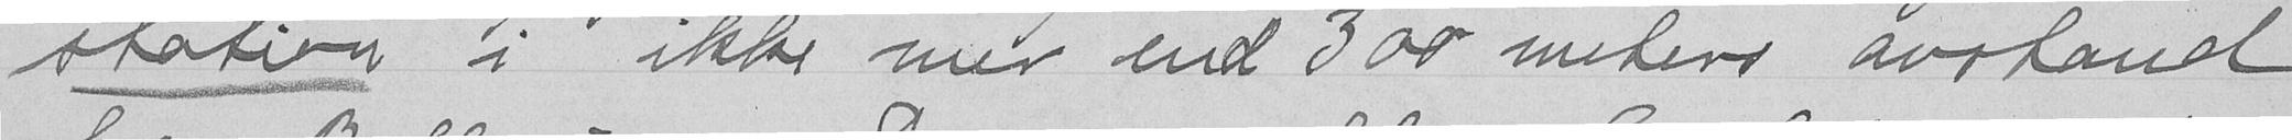station i ikke mer end 300 meters avstand
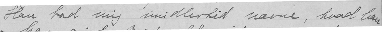Han bad mig imidlertid nævne, hvad han
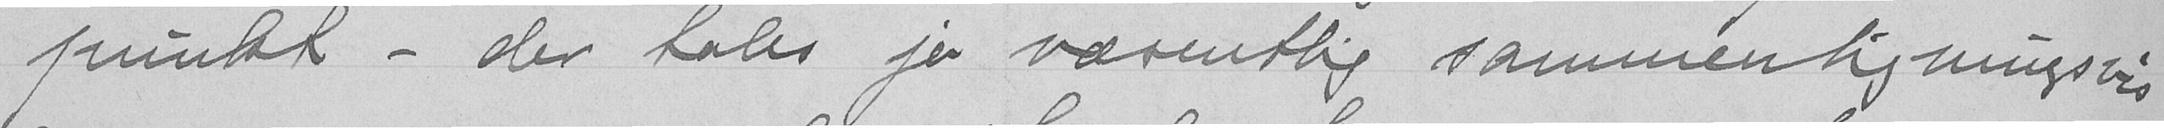punkt - der tales jo væsentlig sammenligningsvis
